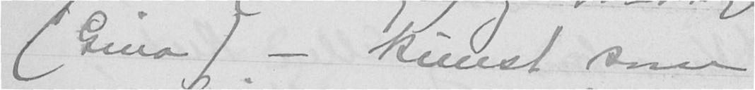(Gina) - kunst som
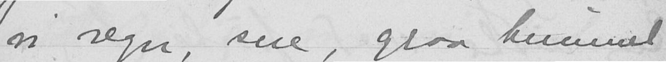vi regn, sne, graa himmel
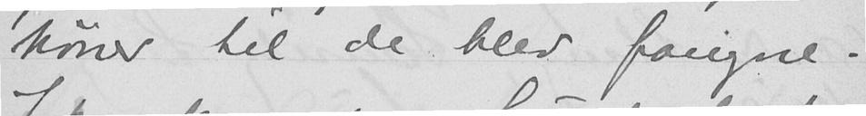høner til de blev fangne.
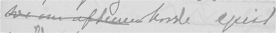her om aftenen havde spist
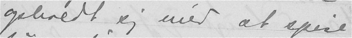opholdt sig med at spise
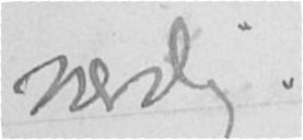værdig.
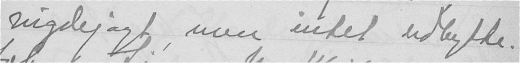rugdejagt, men intet udbytte.
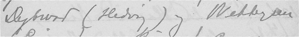Dybwad (Hedvig) og Wettergreen
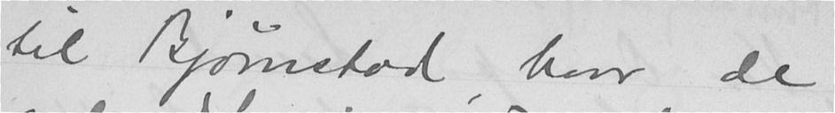til Bjørnstad hvor de
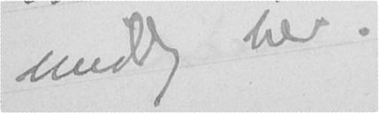middag her.
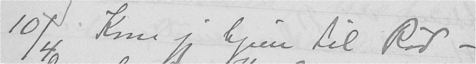10/4 Kom jeg hjem til Rød -
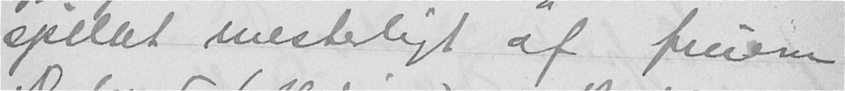spillet mesterligt af fruen
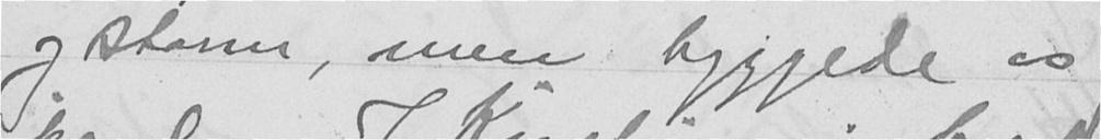og storm, men hyggede os
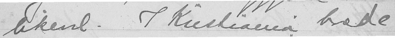likevel. I Kristiania boede
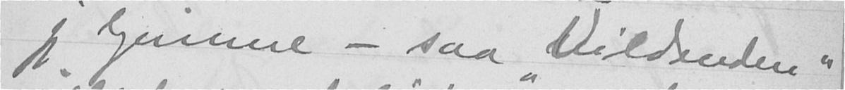jeg hjemme - saa "Vildanden"
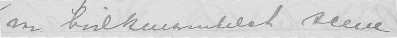var hvilkensomhelst scene
